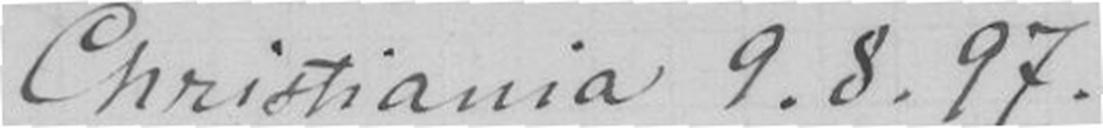Christiania 9.8.97.
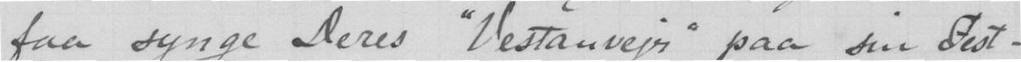faa synge Deres "vestanvejr" paa sin Fest-
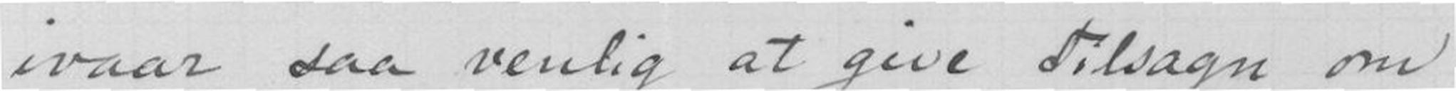ivaar saa venlig at give Rilsagn om,
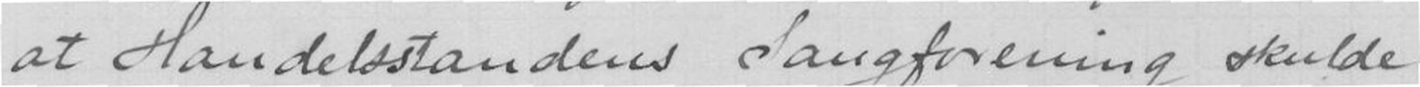at Handelsstandens Sangforening skulde
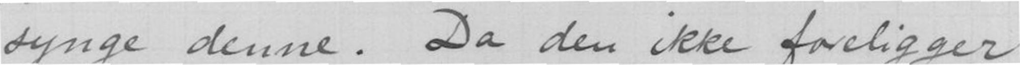synge denne. Da den ikke foreligger
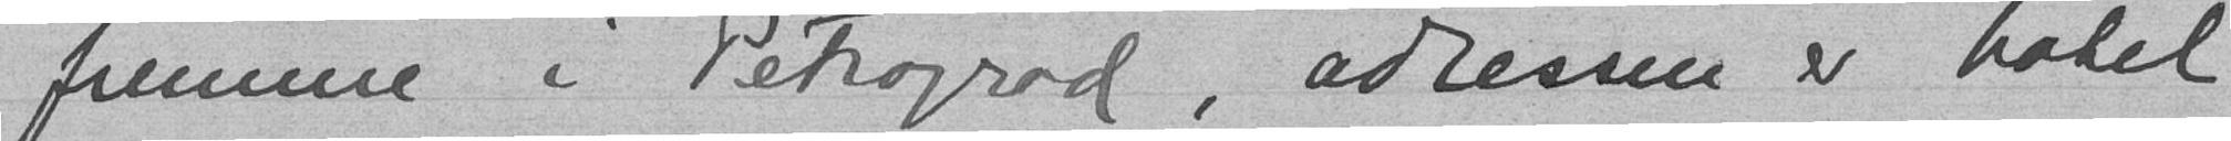fremme i Petrograd, adressen er hotel
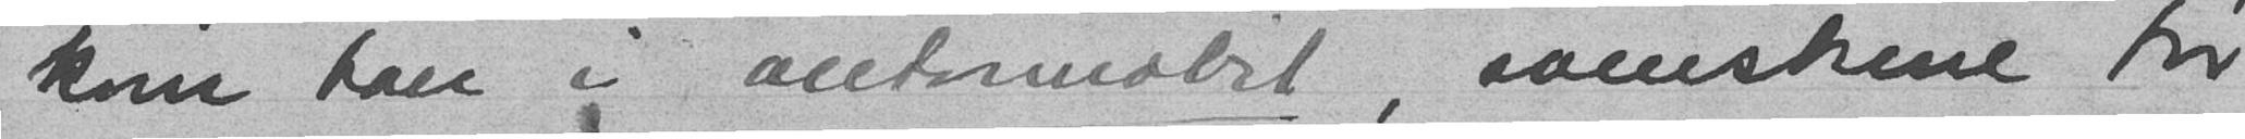kom han i automobil, svenskene tør
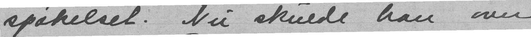spøkelset. Nu skulde han over
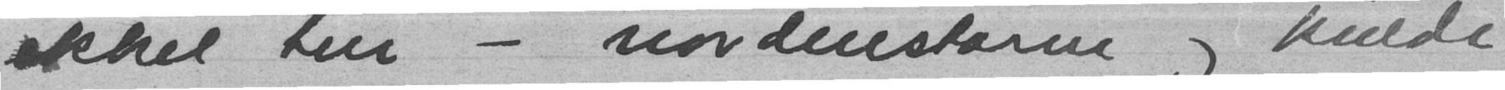ekkel tur - nordenstorm og kulde
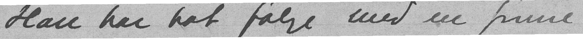Han har hat følge med en finne.
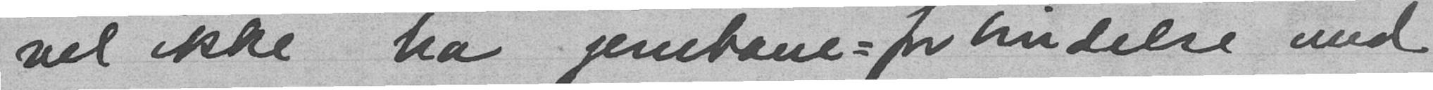vek ikke ha jernbane-forbindelse med
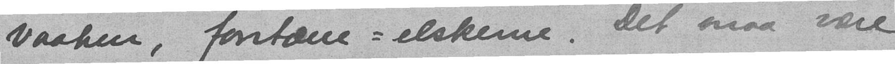vaaben, fontæne-elskerne. Det maa være
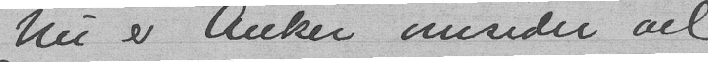Nu er Anker omsider vel
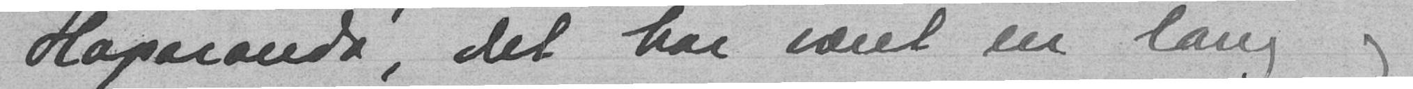Haparanda, det har været en lang og
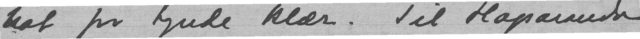hat for tynde klær. Til Harapanda
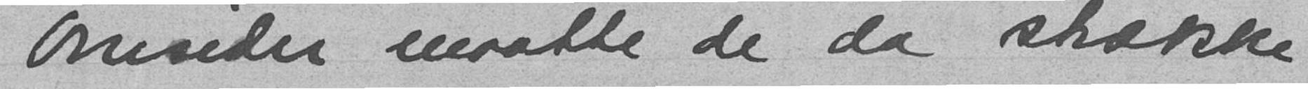Omsider maatte de da strække
